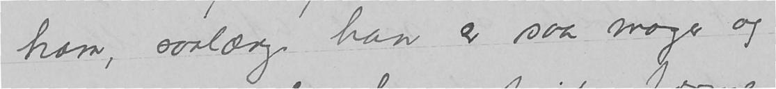ham, saalænge han er saa mager og
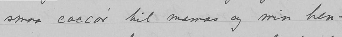smaa caccar til mamas og min hen-
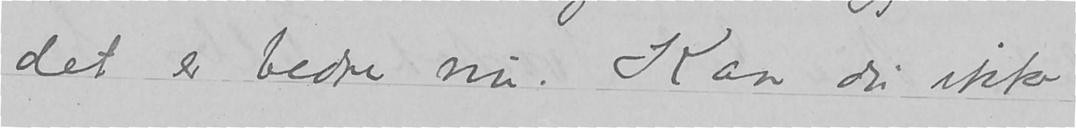det er bedre nu. Kan du ikke
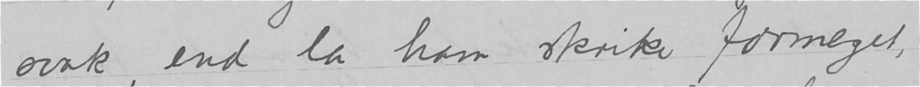svak, end la ham skrike formeget,
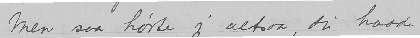Men saa hørte jeg altsaa, du hadde
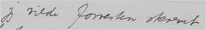jeg vilde forresten skrevet
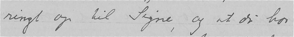ringt op til Signe og at du har
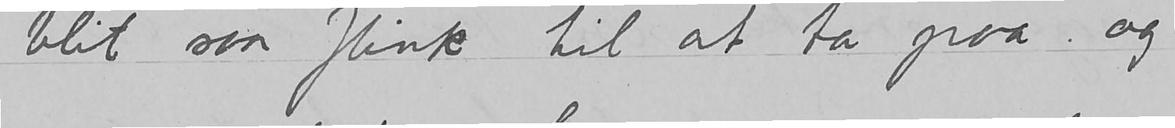blit saa flink til at ta paa og
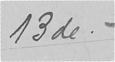13de.
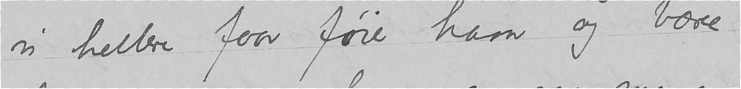vi hellere faar føie ham og bære
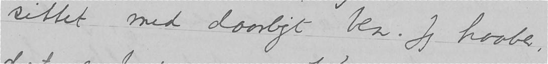sittet med daarligt ben. Jeg haaber,
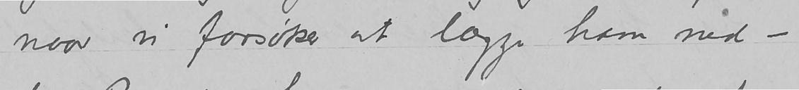naar vi forsøker at lægge ham ned –
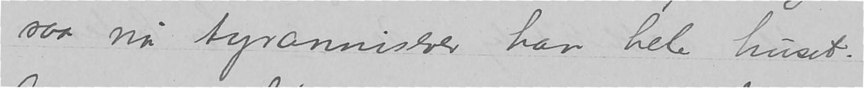saa nu tyranniserer han hele huset.
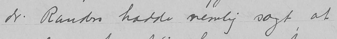dr. Randers hadde nemlig sagt at
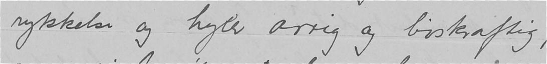rykkelse og hyler arrig og livskraftig,
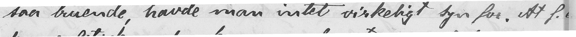saa truende, havde man intet virkeligt syn for. At f. ex.
